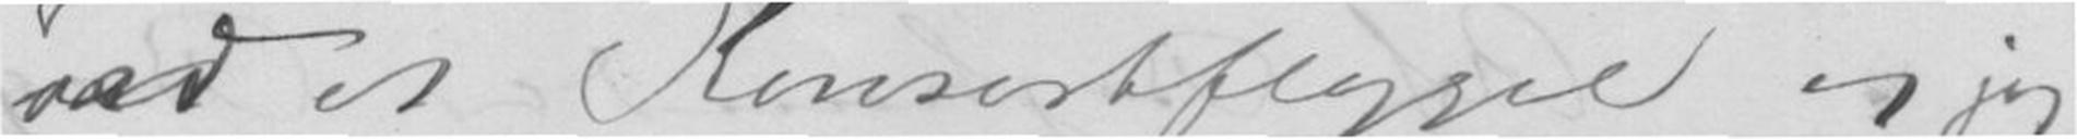ved et Konsertflygel og jeg
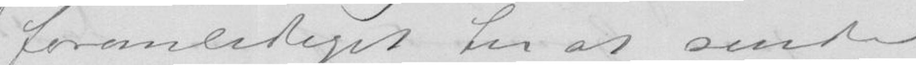foranlediget til at sende
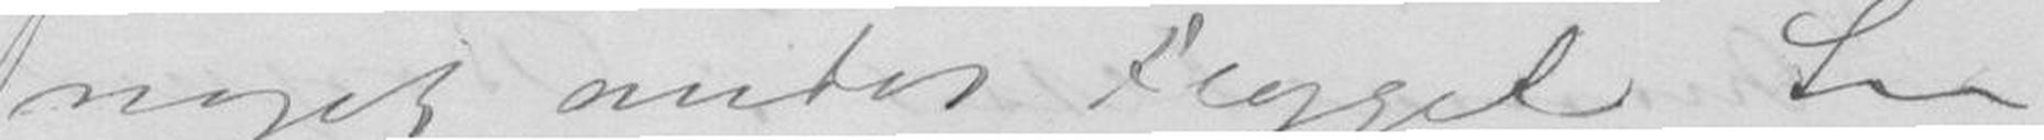noget andet Flygel til
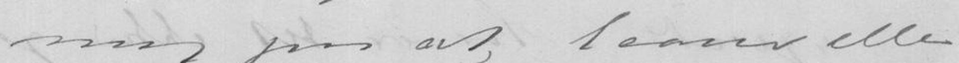mig paa at laane eller
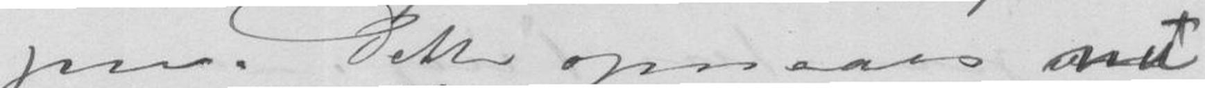paa. Dette opnaaes nu
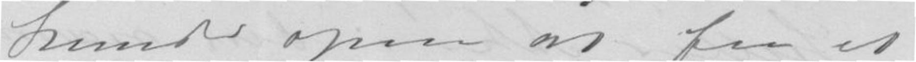kunde opnaa at faa et
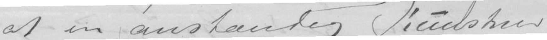at en anstændig Kunstner
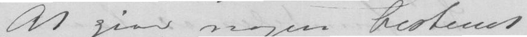At give nogen bestemt
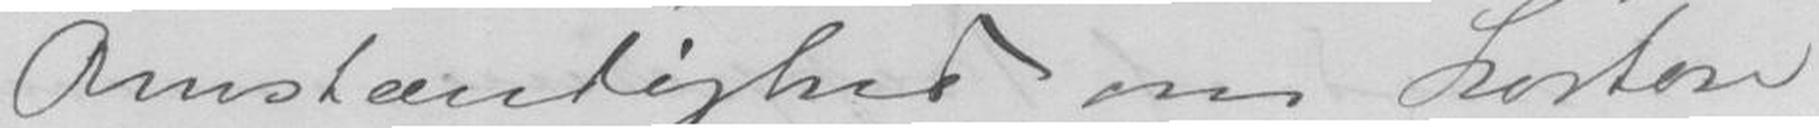Omstændighed om høsten
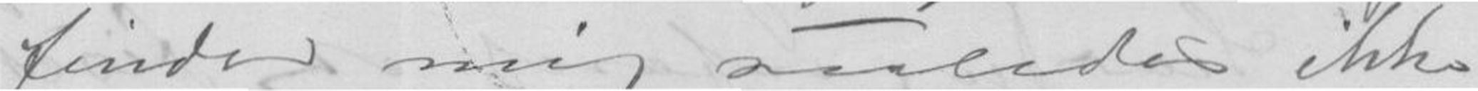finder mig saaledes ikke
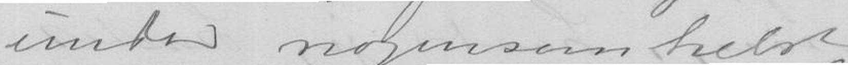under nogensomhelst
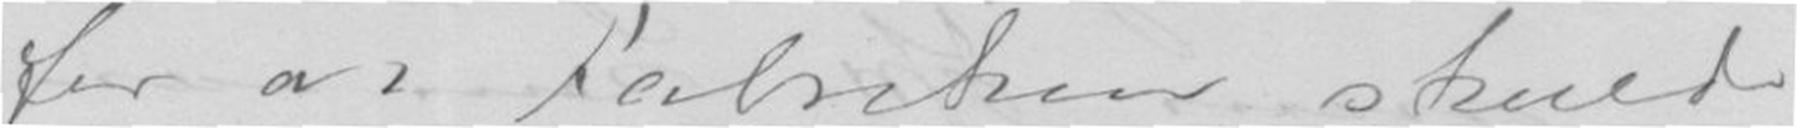for at Fabriken skulde
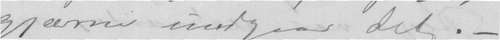gjærne undgaar det. -
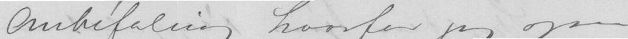Ambefaling hvorfor jeg ogsaa
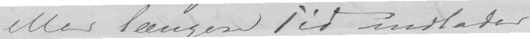eller længere Tid indlader
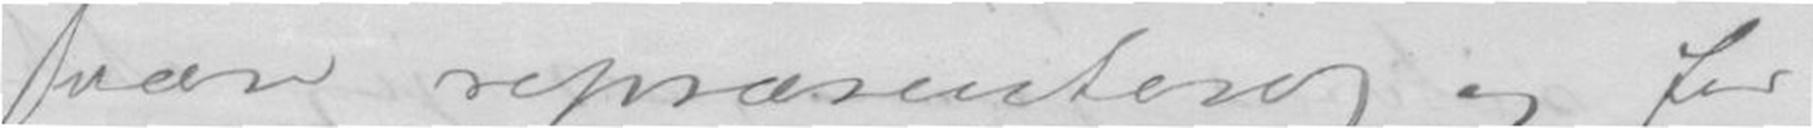være repræsenteret og for
